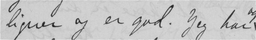ligner og er god. Jeg har
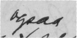ogsaa
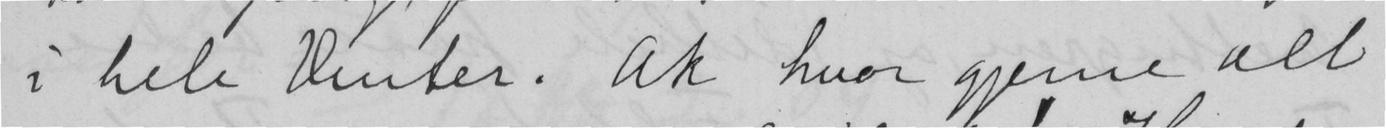i hele Vinter. Ak hvor gjerne alt
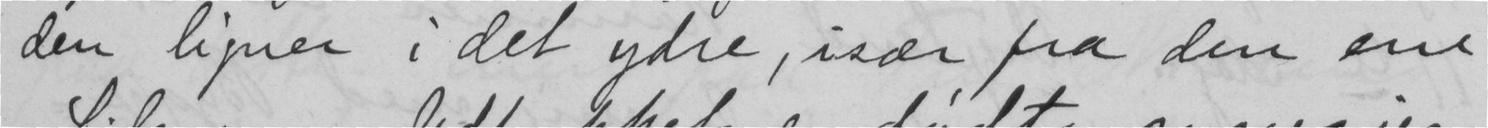den ligner i det ydre, især fra den ene
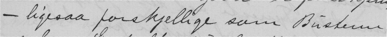- ligesaa forskjellige som Busterne
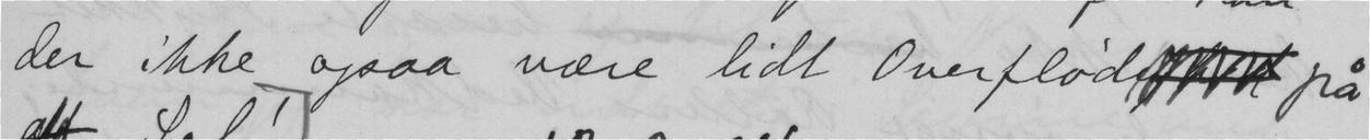der ikke ogsaa være lidt Overflød på
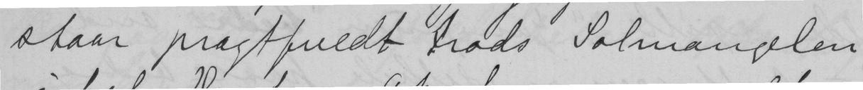staar pragtfuldt trods Solmangelen
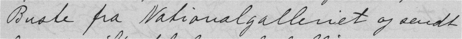Buste fra Nationalgalleriet og sendt
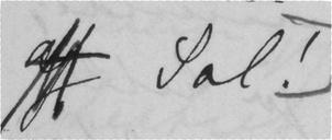Sol!
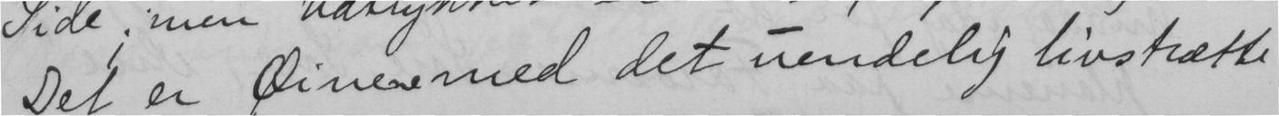Det er Øinene med det uendelig livstrætte
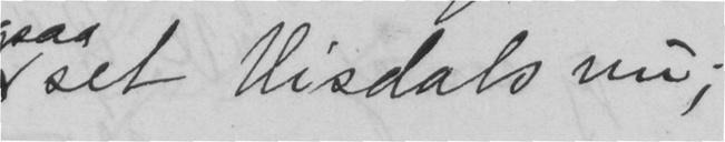set Visdals nu;
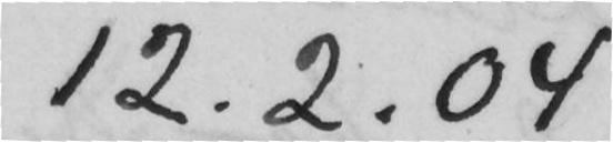12.2.04
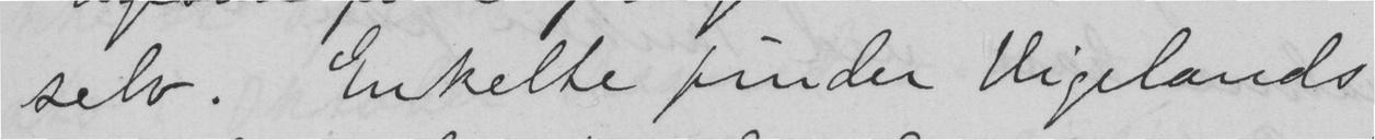selv. Enkelte finder Vigelands
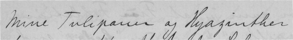Mine Tulipauer og Hyazinther
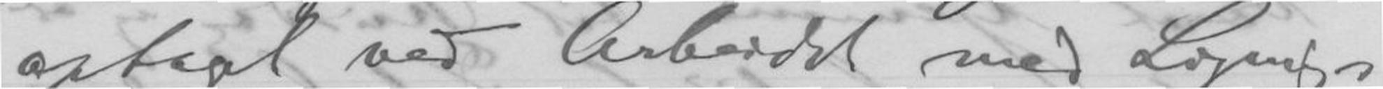optaget ved Arbeidet med Lignings-
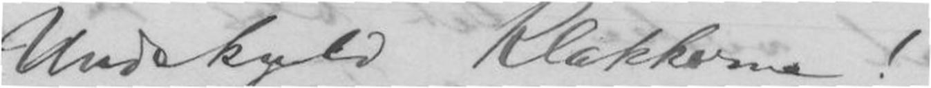Undskyld Klakkerne!
